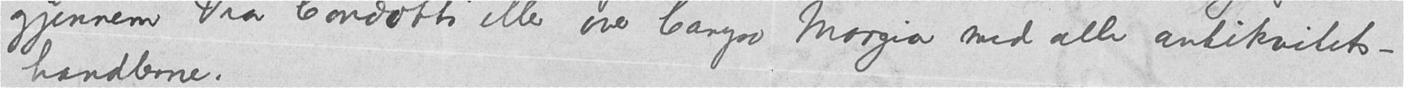gjennem Via Condotti eller over Canyso Marzia med alle antikvitets-
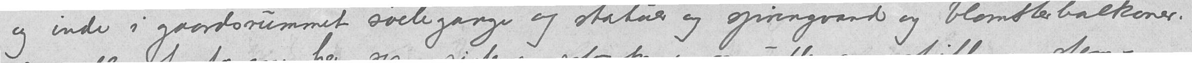og inde i gaardsrummet søileganger og statuer og springvand og blomsterbalkoner.
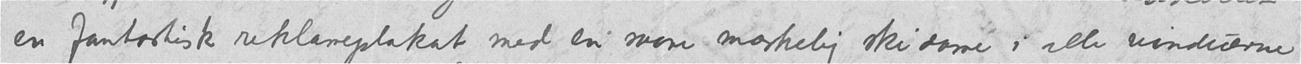en fantastisk reklameplakat med en svare mærkelig skidame i alle vinduerne
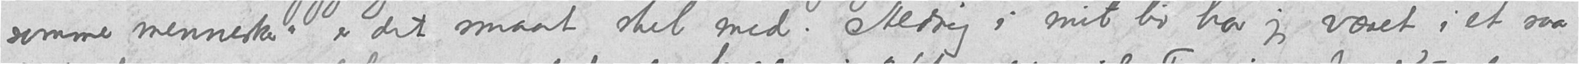somme mennesker" er det smaat stel med. Aldrig i mit liv har jeg været i et saa
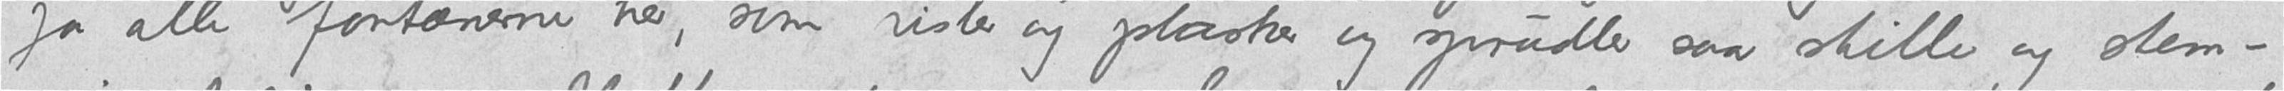Ja alle fontænerne her, som risler og plasker og sprudler saa stille og stem-
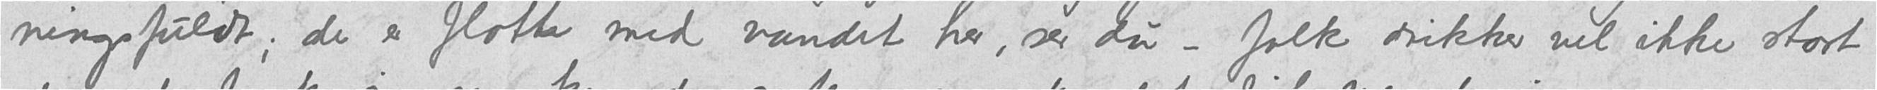ningsfuldt; de er flotte med vandet her, ser du – folk drikker vel ikke stort
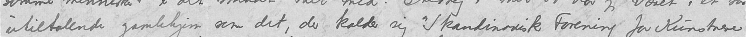utiltalende gamlehjem som det, der kalder sig "Skandinavisk Forening for Kunstnere
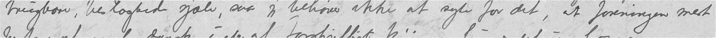brugbare, beslægtede sjæle, saa jeg behøver ikke at syte for det, at foreningen mest
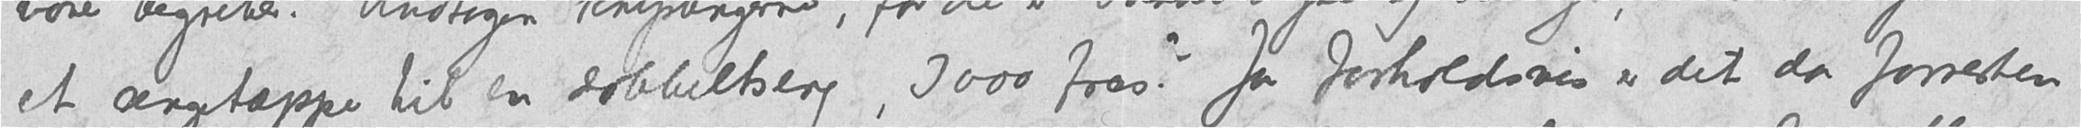et sengetæppe til en dobbeltseng, 3000 fres? Ja forholdsvis er det da forresten
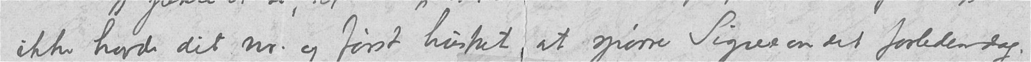ikke havde dit nr. og først husket, at spørre Signe on det forleden dag.
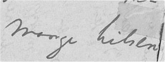Mange hilsen,
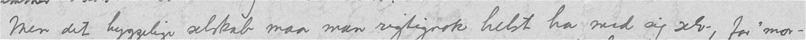Men det hyggelige selskab maa man rigtignok helst ha med sig selv, for "mor-
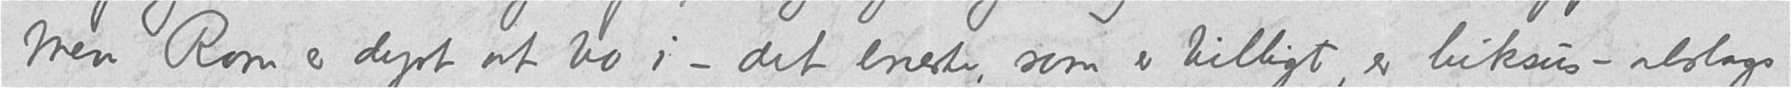Men Rom er dyrt at bo i – det eneste, som er billigt, er luksus - alslags
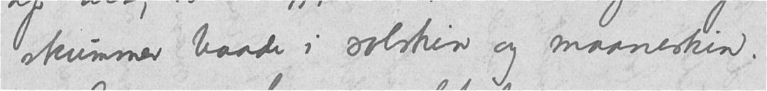skummer baade i solskin og maaneskin.
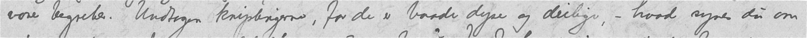vore begreber. Undtagen kniplingerne, for de er baade dyre og deilige, – hvad synes du om
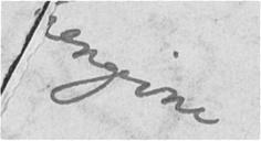hengivne
